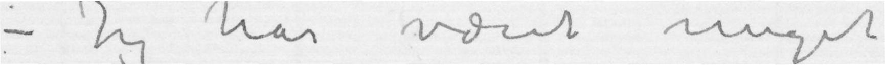– Jeg har været meget
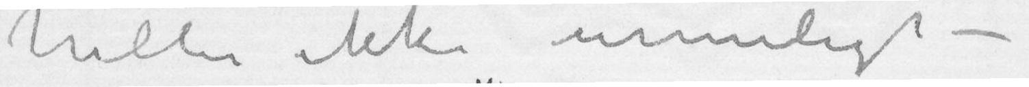heller ikke urimeligt –
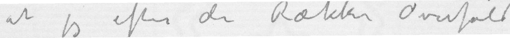at jeg efter de Rækker Overfald
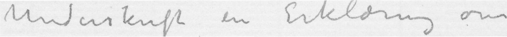Underskrift en Erklæring om
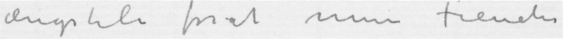ængsteli for at mine Fiender
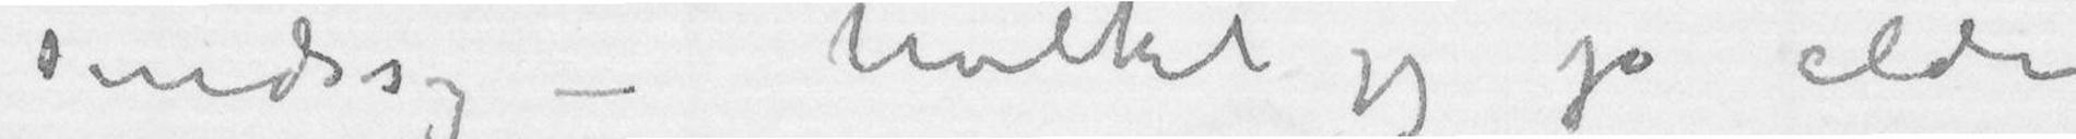sindsyg – hvilket jeg jo aldri
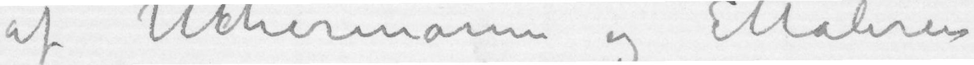af Uchermann og Maleren
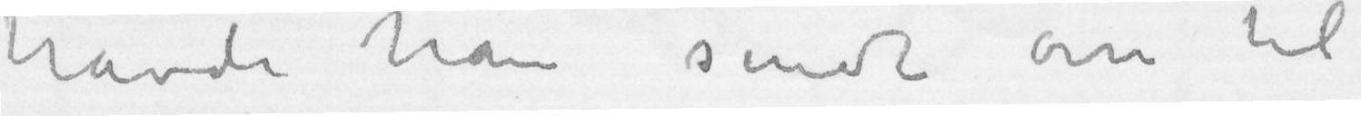havde han sendt om til
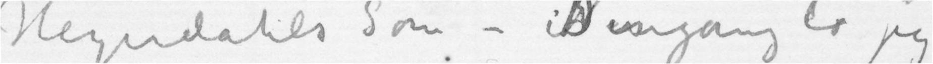Heyerdahls Søn – Dengang lo jeg
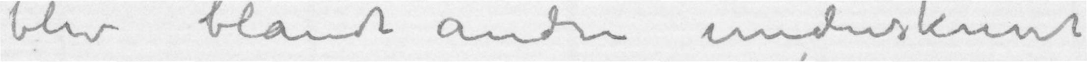blev blandt andre underskrevet
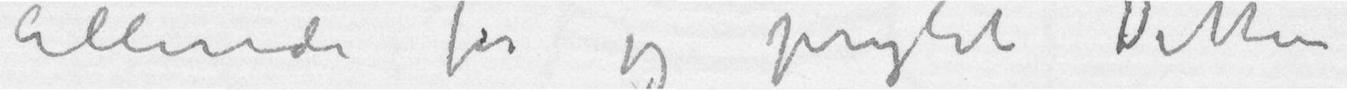Allerede før jeg pryglet Ditten
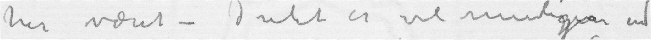har været – Intet er vel rimeligere end
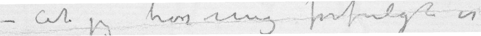– At jeg tror mig forfulgt er
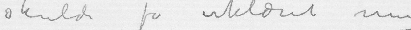skulde få erklæret mig
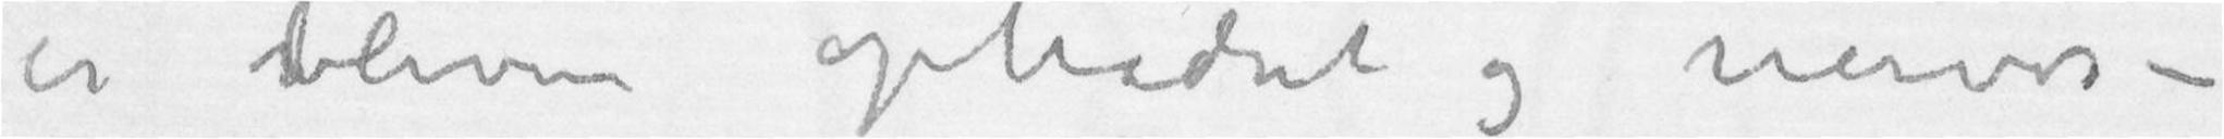er bleven ophidset og nervøs –
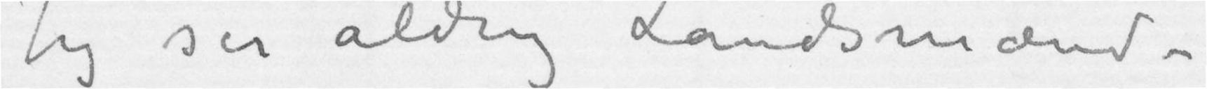Jeg ser aldrig Landsmænd –
